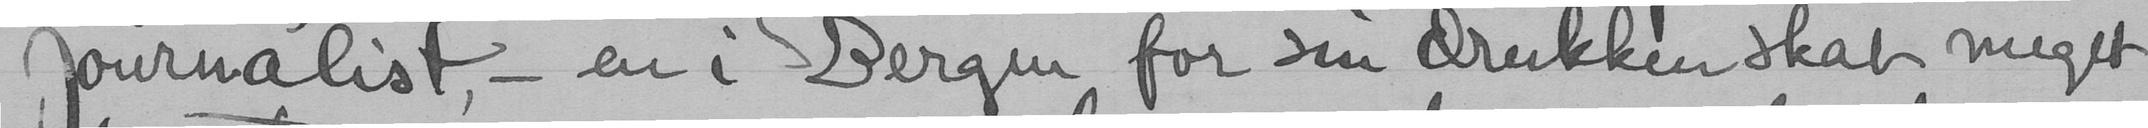journalist, - en i Bergen for sin drukkenskab meget
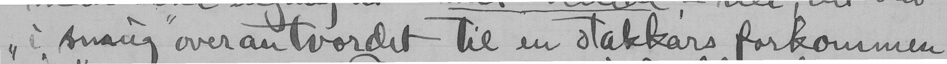"i snaug" overantvordet til en stakkars forkommen
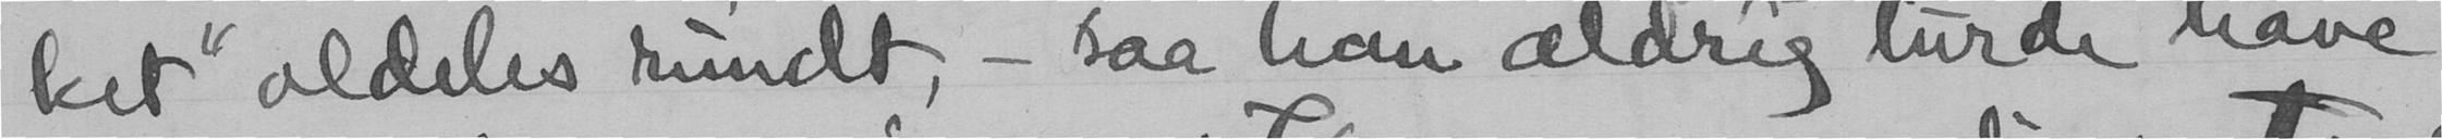ket" aldeles rundt, - saa han aldrig turde have
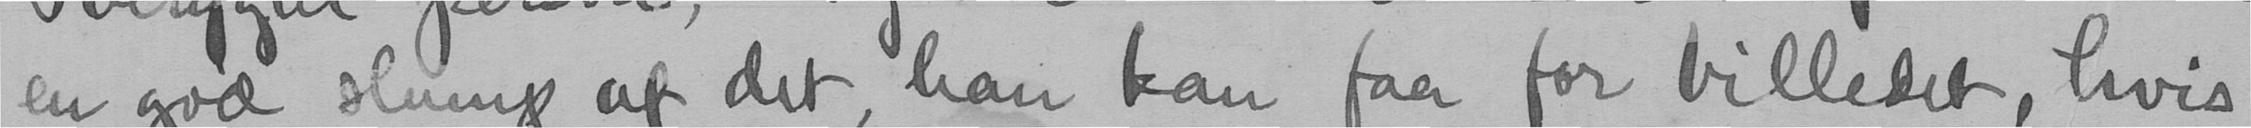en god slump af det han kan faa for billedet, hvis
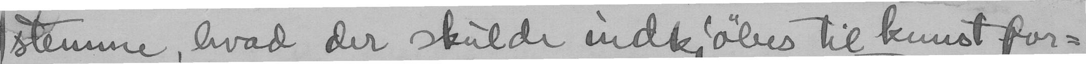stemmne, hvad der skulde indkjøbes til kunst for-
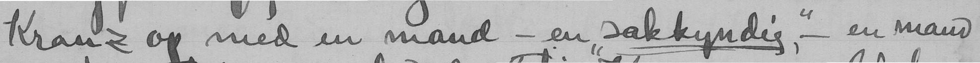Kranz op med en mand - en "sakkyndig", - en mand
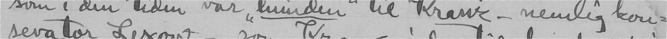som i den tiden var "hunden" til Kranz, - nemlig kon-
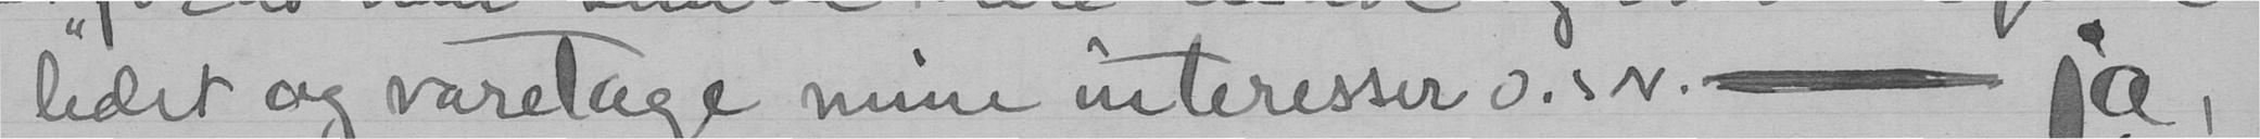ledet og varetage mine interesser o.s.v. - ja,
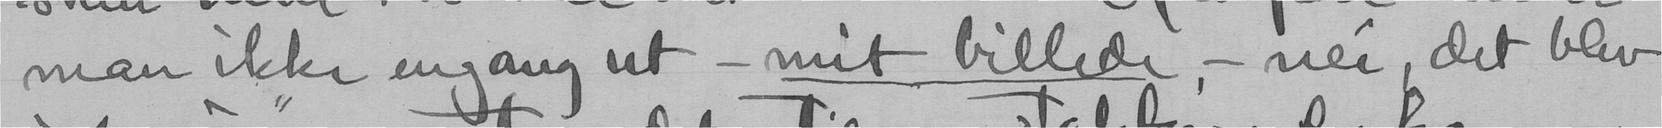man ikke engang uet - mit billede, - nei, det blev
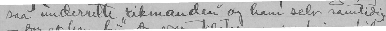saa underrette "rikmanden" og ham selv samtidig,
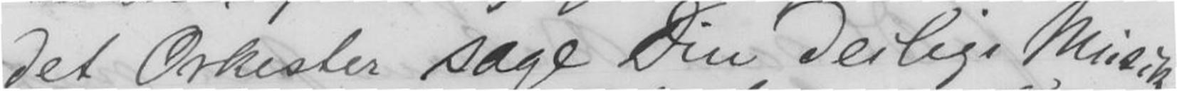det Orkester sage Din deilige Musik
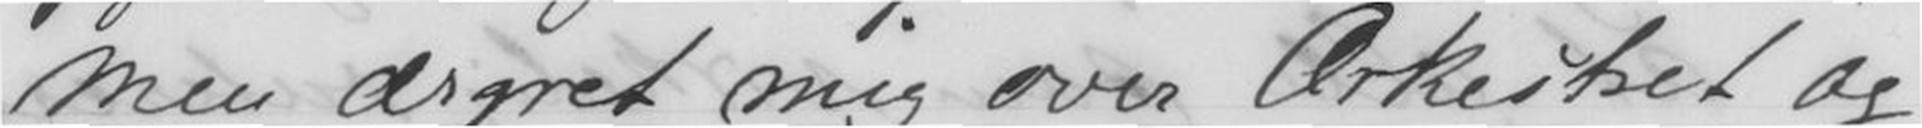men Ærgret mig over Orkestret og
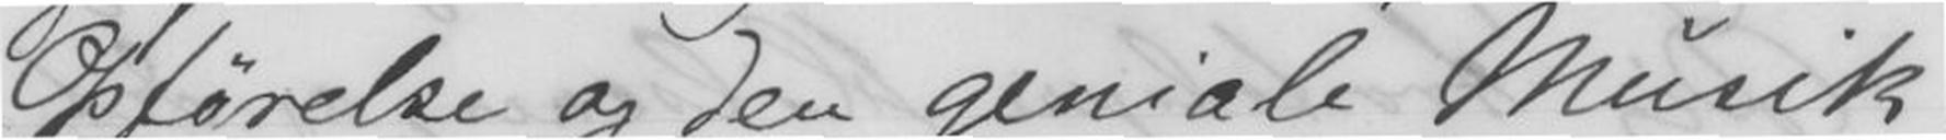Opførelse og den geniale Musik,
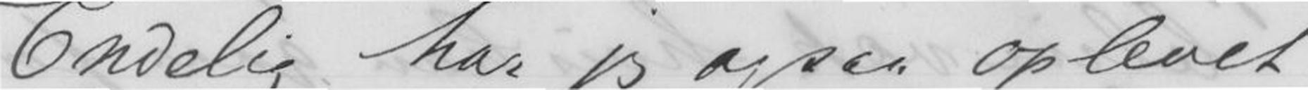Endelig har jeg ogsaa oplevet
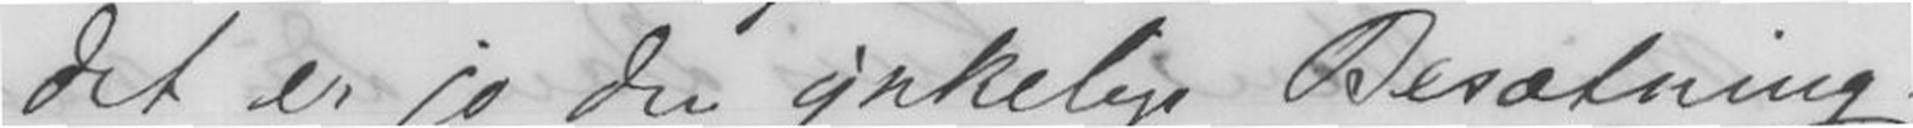det er jo den ynkelige Besætning,
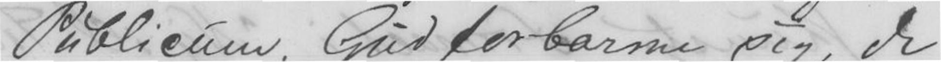Publikum, Gud forbarme sig, de
